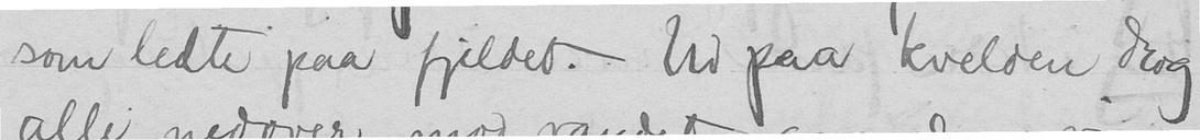som ledte paa fjeldet. Ud paa kvelden drog
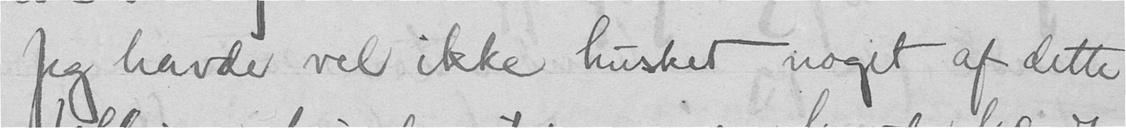Jeg havde vel ikke husket noget af dette
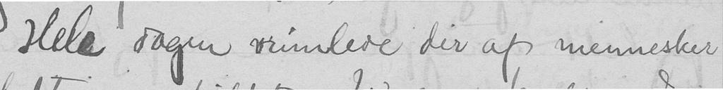Hele dagen vrimlede der af mennesker
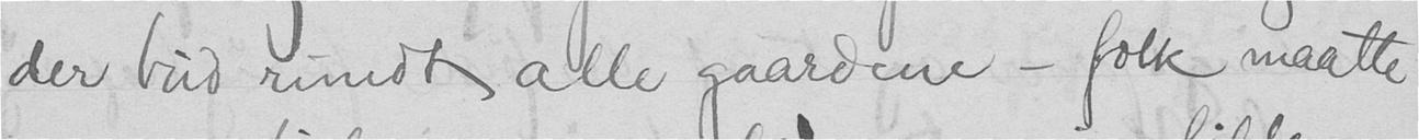der bud rundt alle gaardene - folk maatte
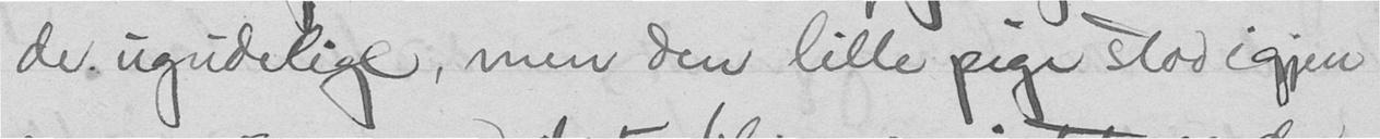de ugudelige, men den lille pige stod igjen
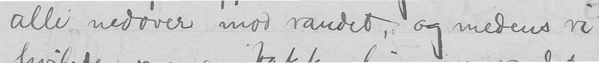alle nedover mod vandet, og medens vi
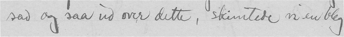sad og saa ud over dette, skimtede vi en bleg
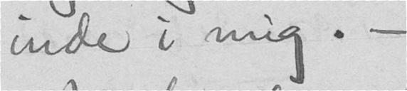inde i mig. -
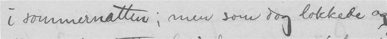i sommernatten; men som dog lokkede og
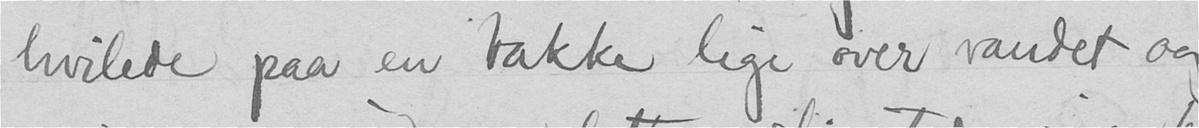hvilede paa en bakke lige over vandet og
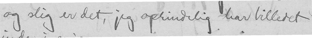og slig er det, jeg oprindelig har billedet
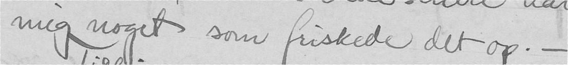mig noget som friskede det op. -
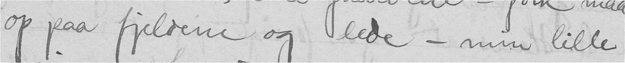op paa fjeldene og lede - min lille
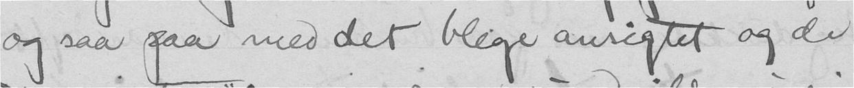og saa paa med det blege ansigtet og de
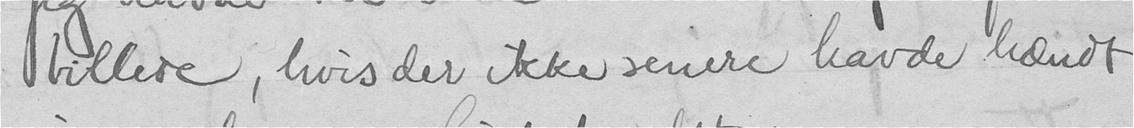billede, hvis der ikke senere havde hændt
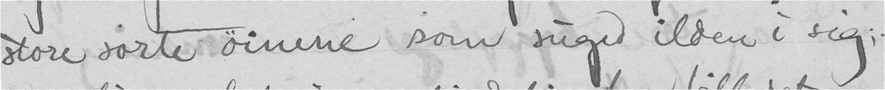store sorte øinene som suged ilden i sig;-
